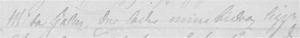Winter Hjelm, der lader mine Bidrag ligge
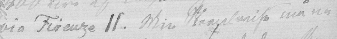via Firenze 11. Min Neapelreise må na
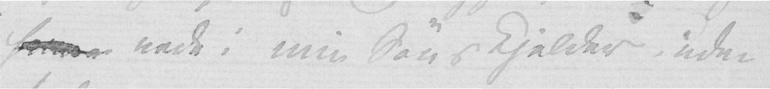henne nede i min Søns kjelder, uden
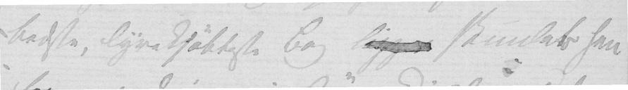bedste, dyrekjøbteste Bog ligger skindet hen
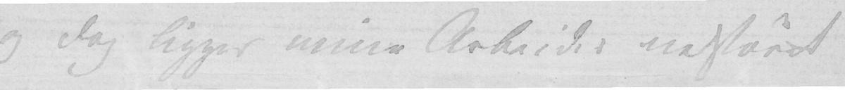og dog ligger mine Arbeider nedstøvet
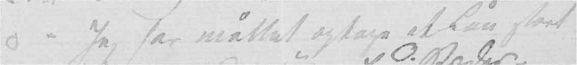Jeg har måttet optage at Lån stort
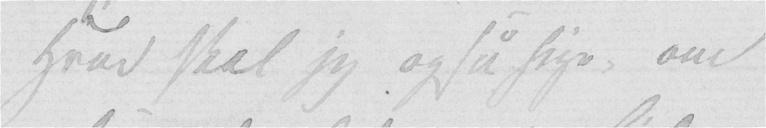Hvad skal jeg også sige, om
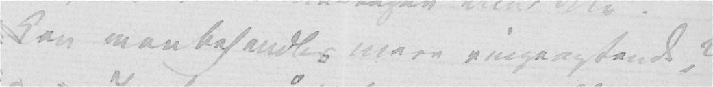Kan man behandles mere ringeagtende?
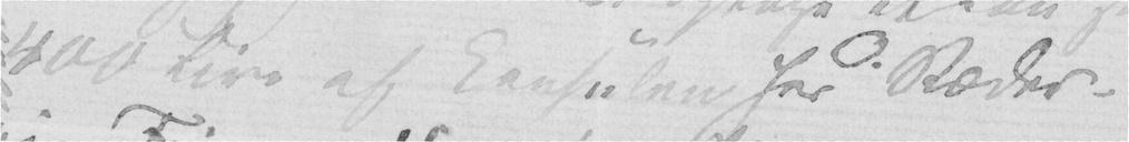400 Lire af Konsulen her O. Ræder.
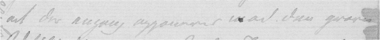at der engang opponeres mod den grove
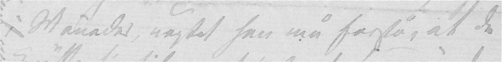i Måneder, uagtet han må forstå, at de
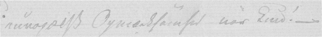annogalsk Opmærksomhed når kun! –
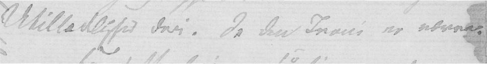Utilladlighed deri. Se den Ironi er værre.
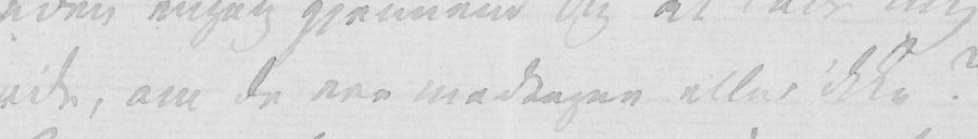vide, om de ere modtagne eller ikke?
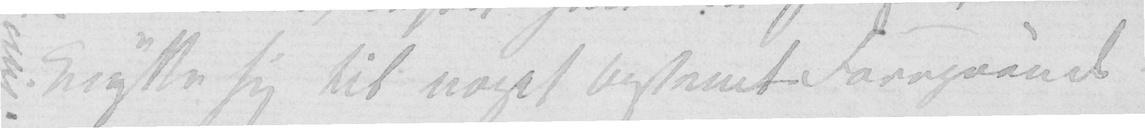knytte sig til noget bestemt Foregaende
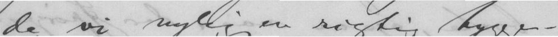de vi nylig en rigtig hygge-
